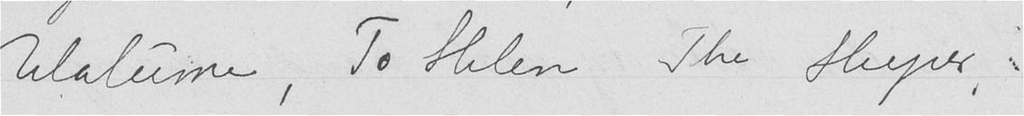Ulalume, To Helen, The Sleeper,
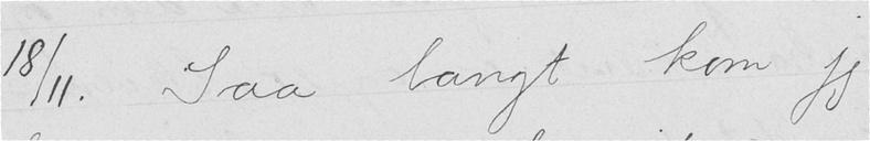18/11. Saa langt kom jeg
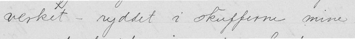verket - ryddet i skufferne mine
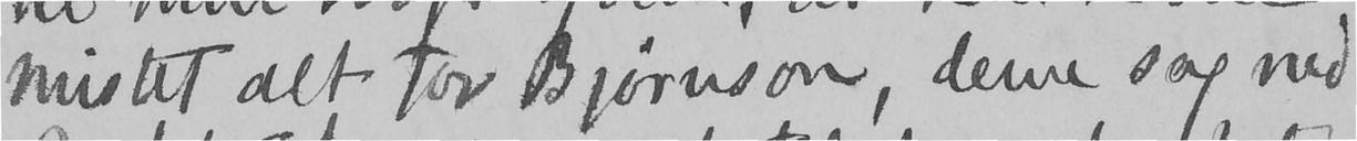mistet alt for Bjørnson, denne sag med
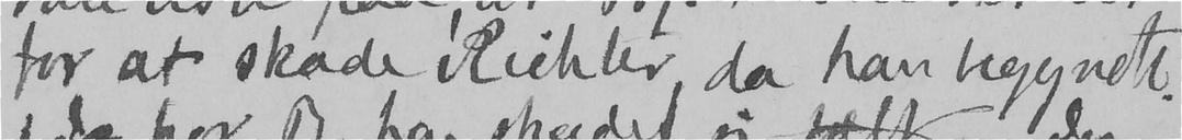for at skade Richter, da han begyndte.
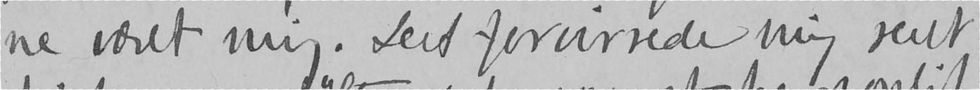ne været mig. Det forvirrede mig rent
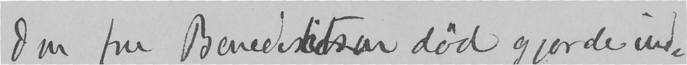Om fru Benediktsen død gjorde ind-
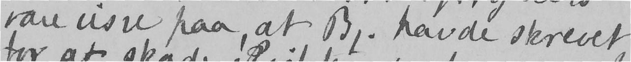vare visse paa, at B,. havde skrevet
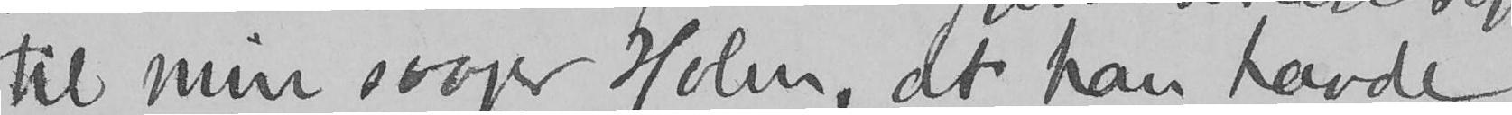til min svoger Holm, at han havde
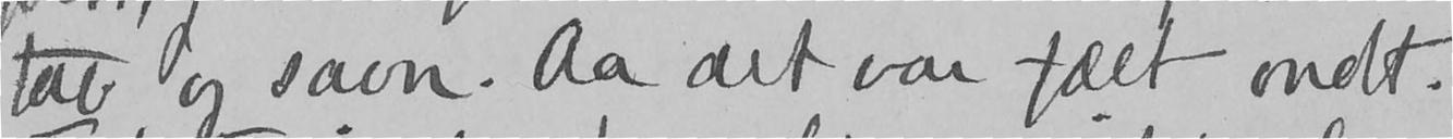tab og savn. Aa det var fælt ondt.
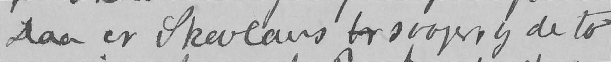Daa er Skavlans bor svoger, og de to
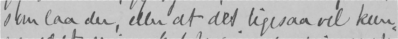som laa der, eller at det ligesaa vel kun-
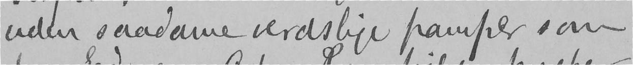uden saadanne verdslige pamper som
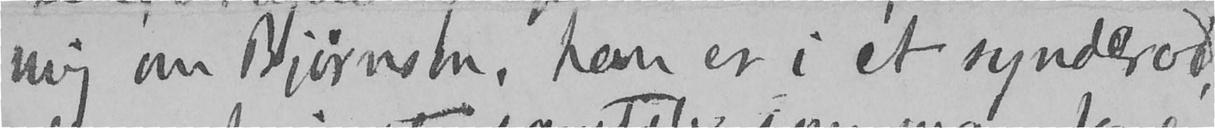mig om Bjørnson, han er i et synderod,
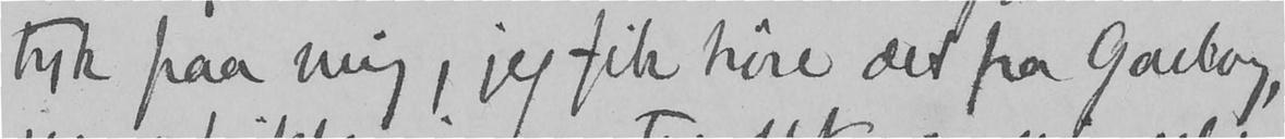tryk paa mig, jeg fik høre det fra Garborg,
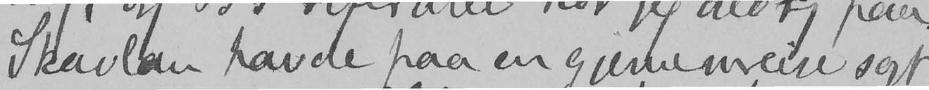Skavlan havde paa en gjennemreise sagt
